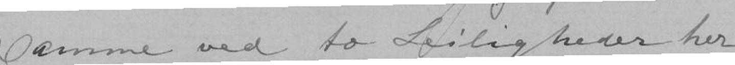samme ved to Leiligheder her
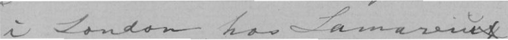i London, hos Lamaviut
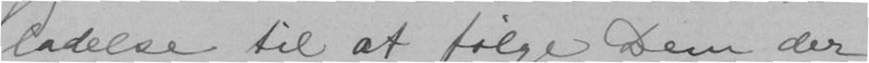ladelse til at følge Dem der
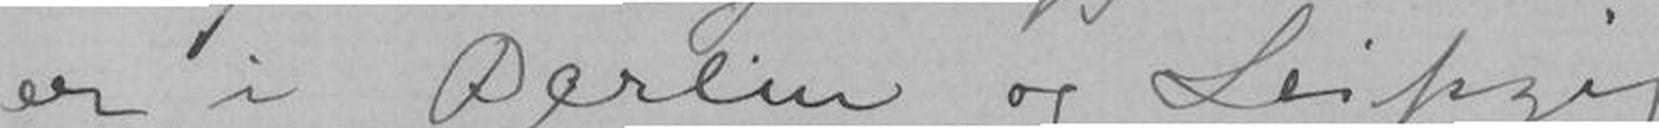er i Berlin og Leipzig,
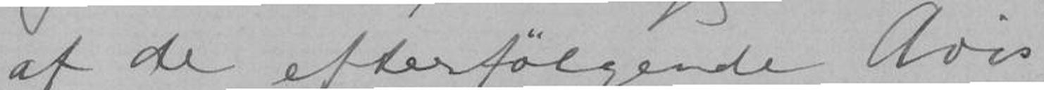at de efterfølgende Avis
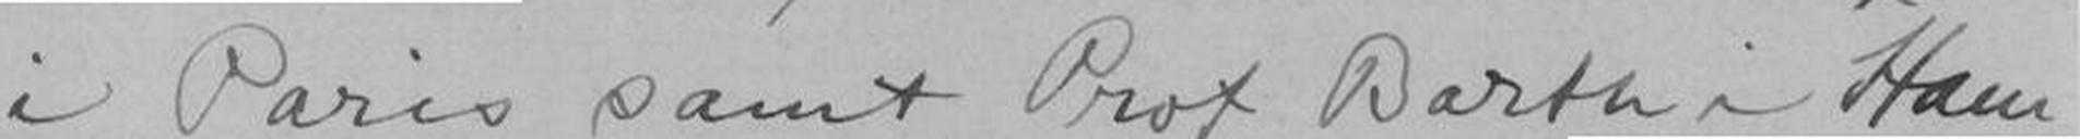i Paris samt Prof Barth i Ham-
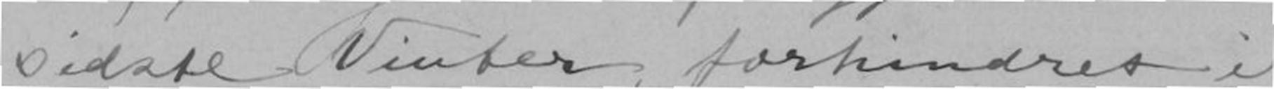sidste Vinter, forhindret i
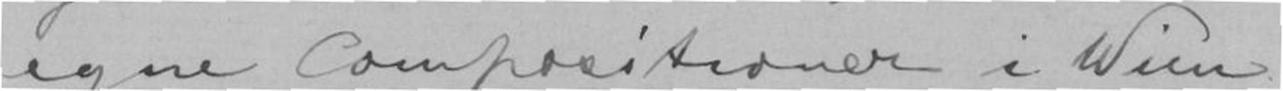egne Compositioner i Wien;
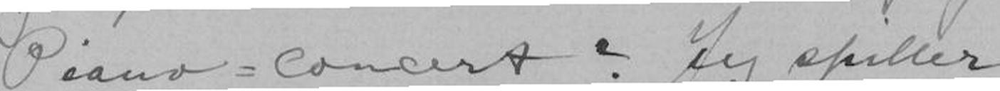Piano=Concert? Jeg spiller
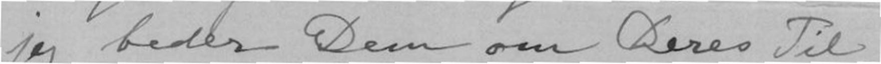jeg beder Dem om Deres Til-
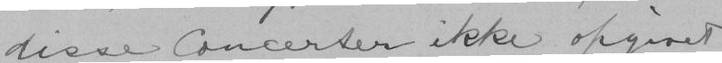disse Concerter ikke opgivet
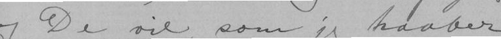og De vil, som jeg haaber
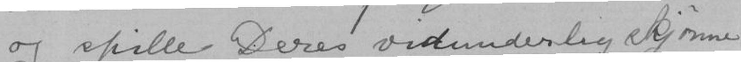og spille Deres vidunderlige skjønne
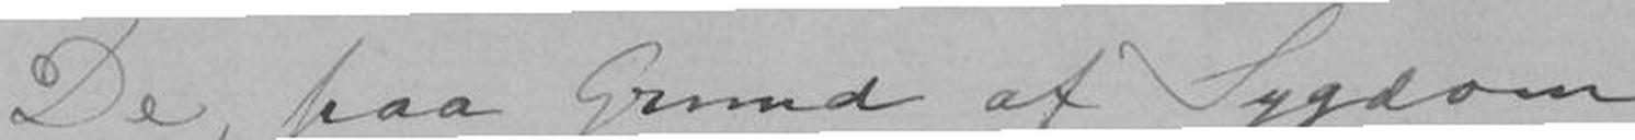De, paa Grund af Sygdom
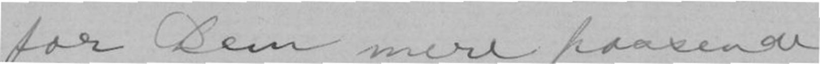for Dem mere passende
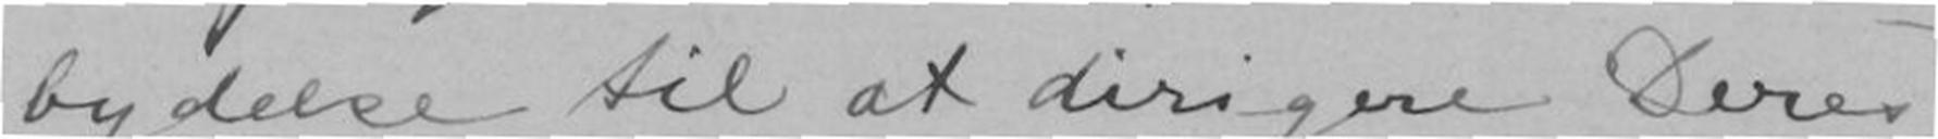bydelse til at dirigere Deres
